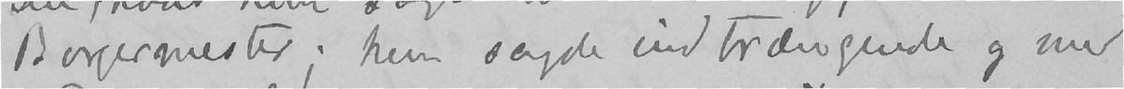Borgermester; hun sagde indtrængende og med
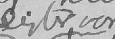digter var
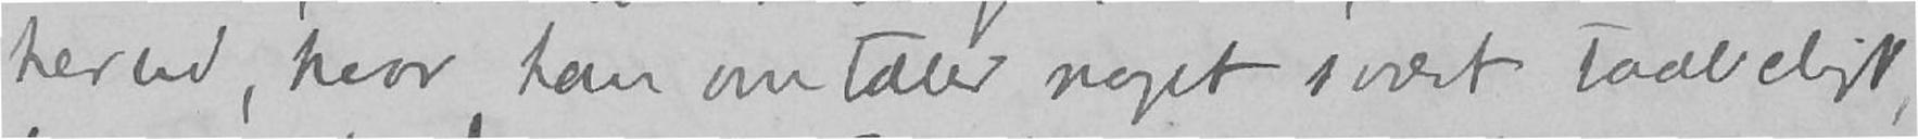herved, hvor han omtaler noget svært taabeligt,
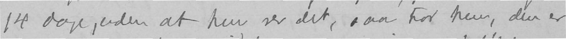14 dage, uden at hun ser det, saa tror hun, den er
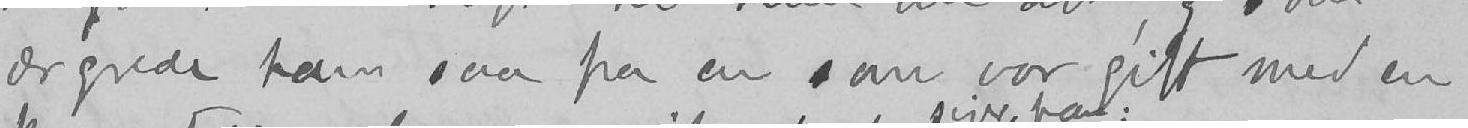ærgrede ham saa fra en som var gift med en
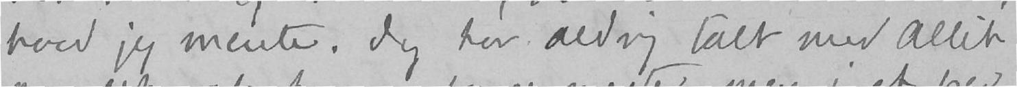hvad jeg mente. Jeg har aldrig talt med Allik
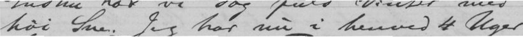Høi Sne. Jeg har nu i henved 4 Uger
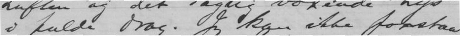i fulde drag. Jeg kan ikke forstaa,
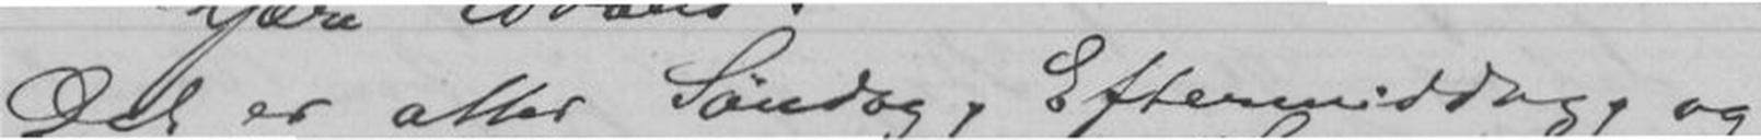Det er atter Søndag, Eftermiddag, og
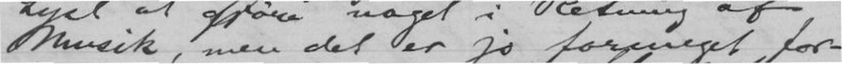Musik, men det er jo formeget for-
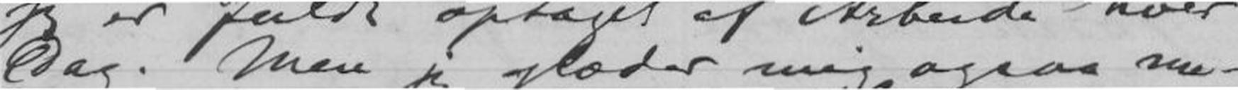Dag. Men jeg glæder mig ogsaa me-
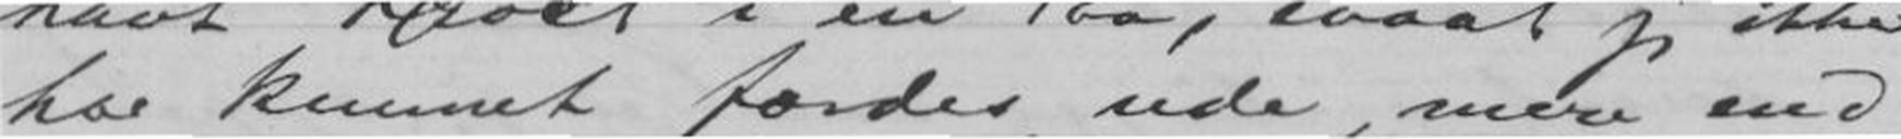har kunnet færdes ude mere end
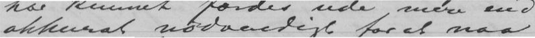akkurat nødvendigt for at naa
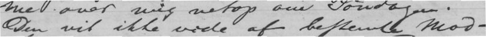Den vil ikke vide af bestemte Mod-
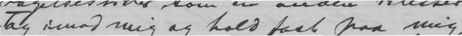Tag imod mig og hold fast paa mig,
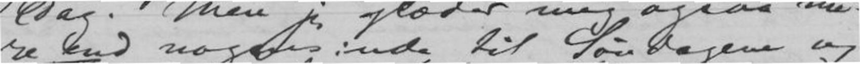re end nogensinde til Søndagene og
